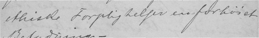ethiske Forpligtelser en forhøiet
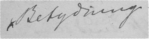Betydning
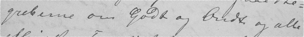greberne om Godt og Ondt og alle
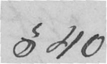§ 40
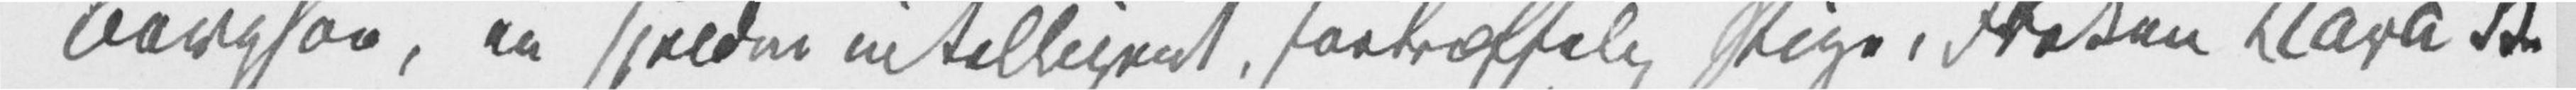Bergsøe, en sjelden intelligent, fortræffelig Pige, Frøken Clara B.
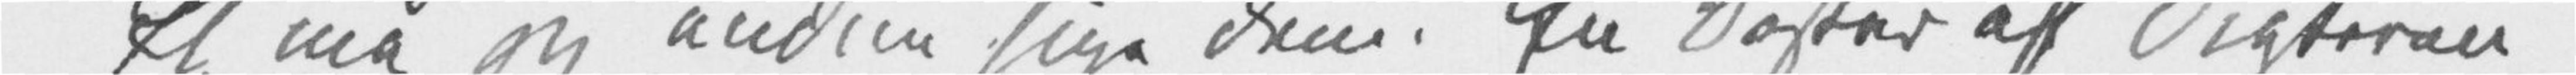Et må jeg endnu sige Dem. En Søster af Digteren
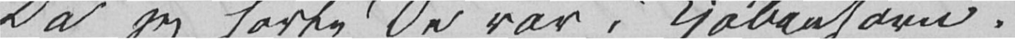Da jeg hørte De var i Kjøbenhavn,
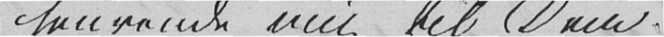henvende mig til Dem.
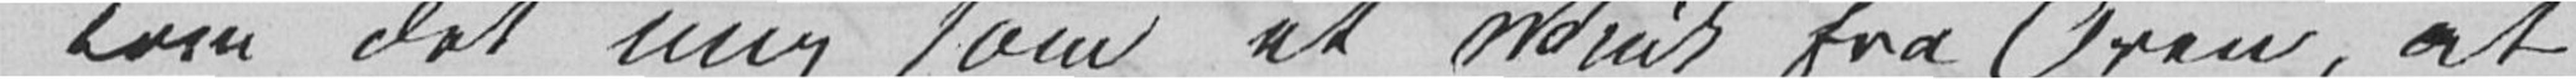kom det mig som et Vink fra Oven, at
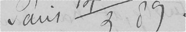Paris 14/3 89.
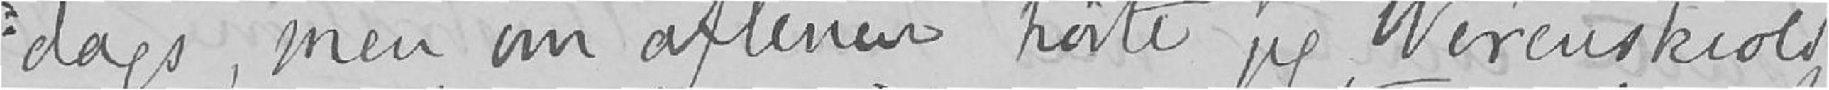dags, men om aftenen hørte jeg Werenskiold
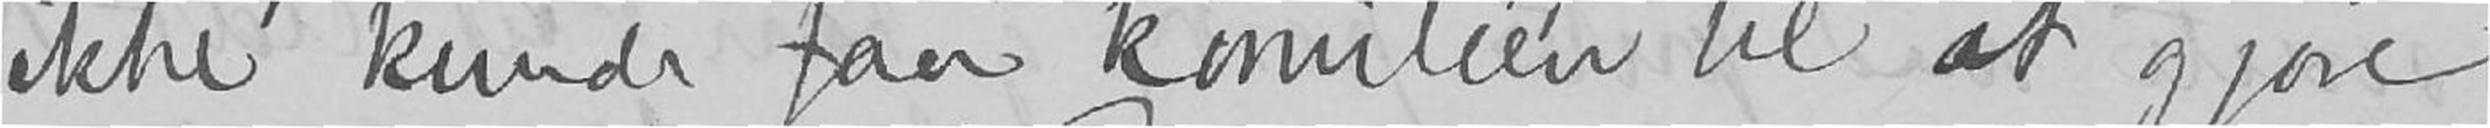ikke kunde faa komitéen til at gjøre
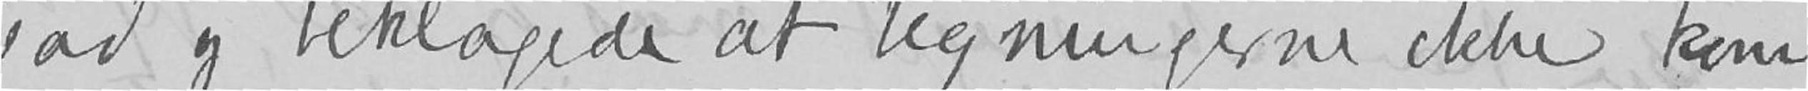sad og beklagede at tegningerne ikke kom
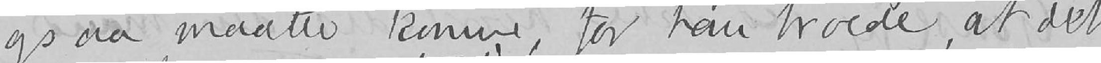ogsaa maatte komme, for han troede, at det
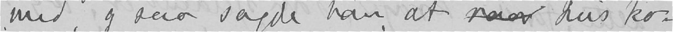med, og saa sagde han, at naar hvis ko-
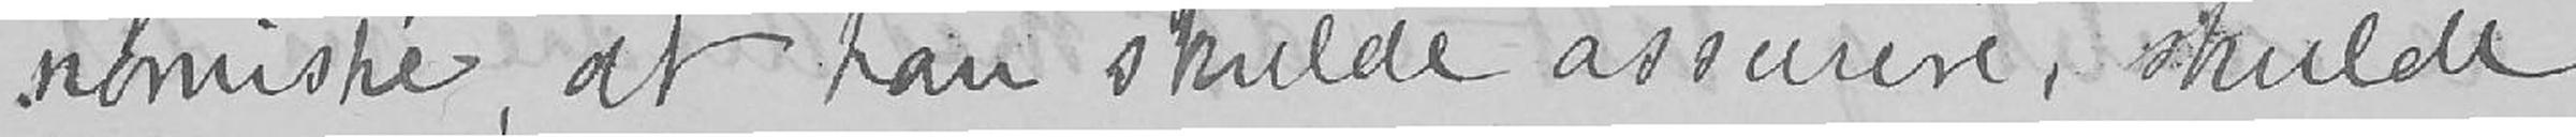nomiske, at han skulde assurere, skulde
